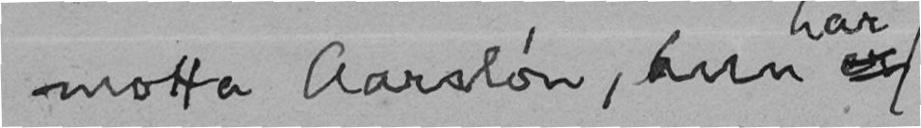motta Aarsløn, hun er
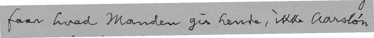faar hvad Manden gir hende, ikke Aarsløn
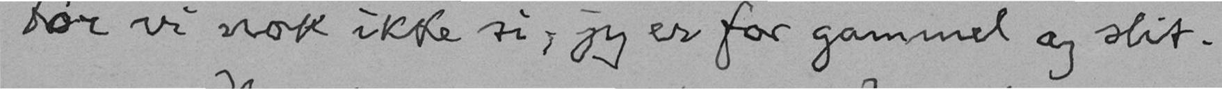tør vi nok ikke si, jeg er for gammel og slit.
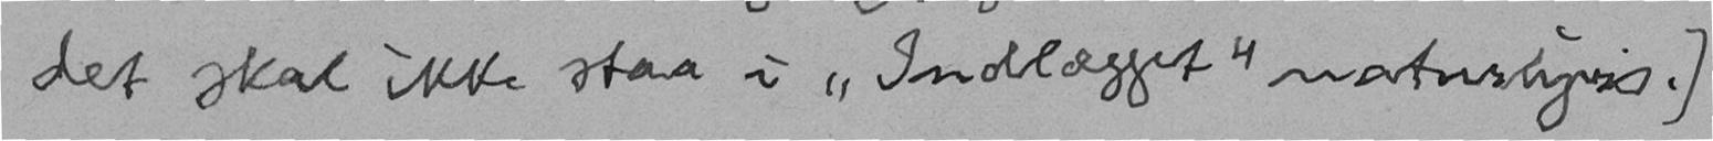det skal ikke staa i "Indlægget" naturligvis.)
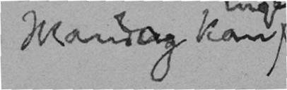Mandag kan
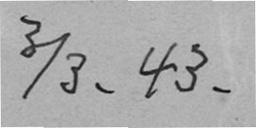3/3. 43.
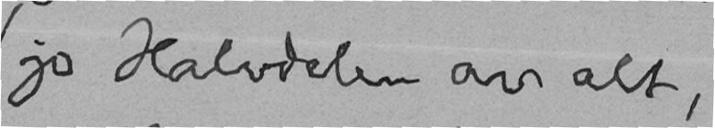jo Halvdelen av alt,
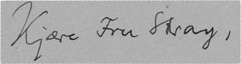Kjære Fru Stray,
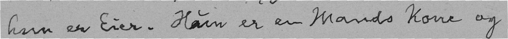hun er Eier. Hun er en Mands Kone og
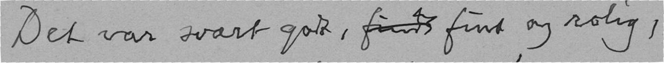Det var svært godt, findt fint og rolig,
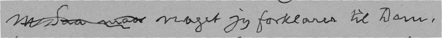m Saa maa noget jeg forklarer til Dem,
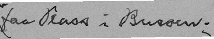faa Plass i Bussen.
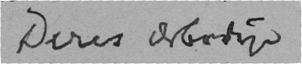Deres ærbødige
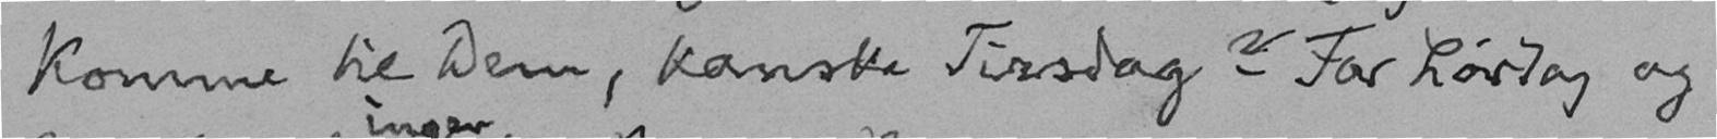komme til Dem, kanske Tirsdag? For Lørdag og
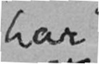har
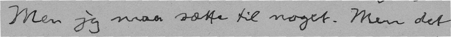Men jeg maa sætte til noget. Men det
